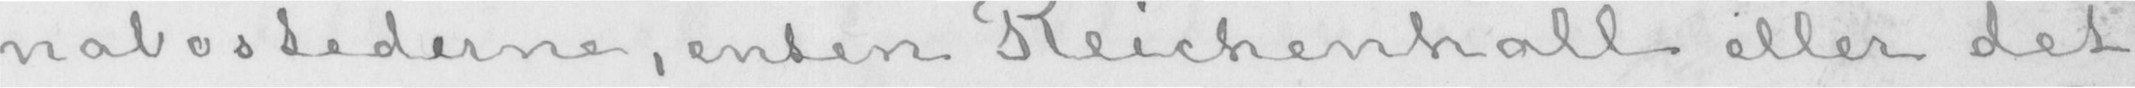nabostederne, enten Reichenhall eller det
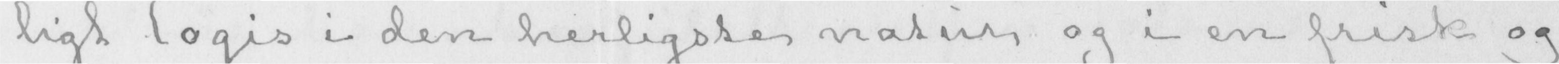ligt logis i den herligste natur og i en frisk og
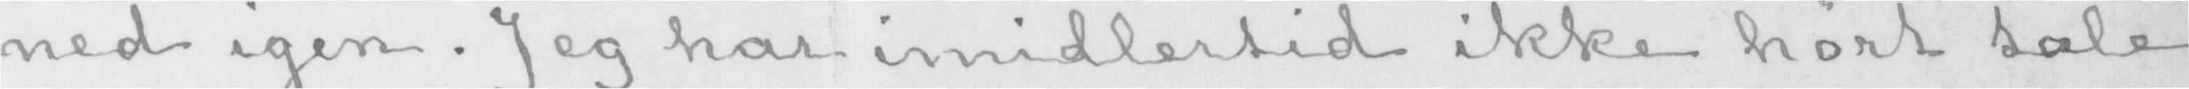ned igen. Jeg har imidlertid ikke hørt tale
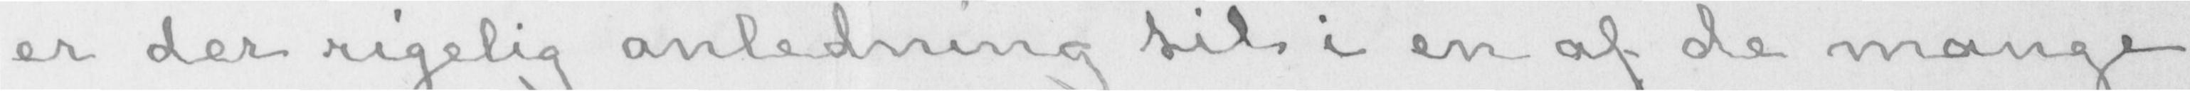er der rigelig anledning til i en af de mange
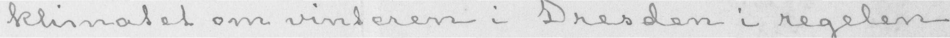klimatet om vinteren i Dresden i regelen
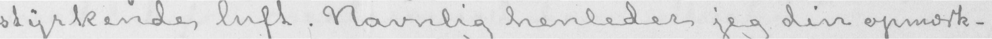styrkende luft. Navnlig henleder jeg din opmærk-
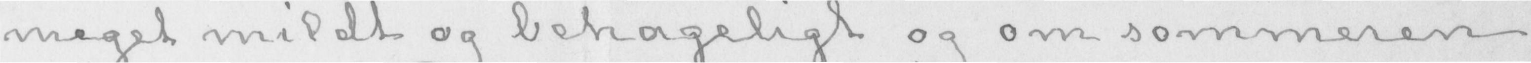meget mildt og behageligt og om sommeren
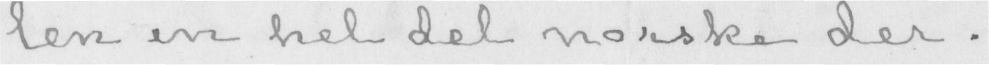len en hel del norske der.
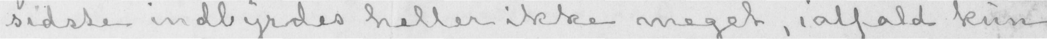sidste indbyrdes heller ikke meget, ialfald kun
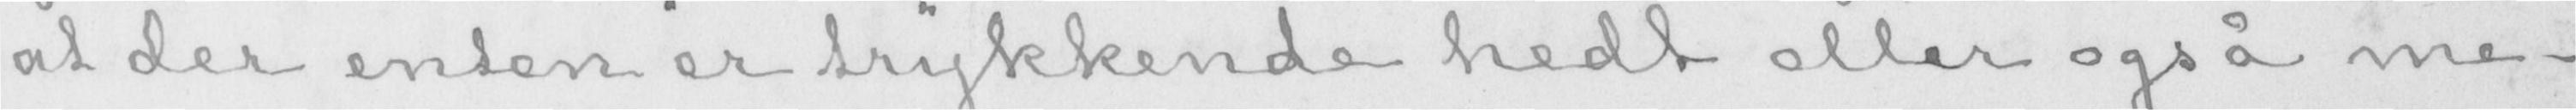at der enten er trykkende hedt eller også me-
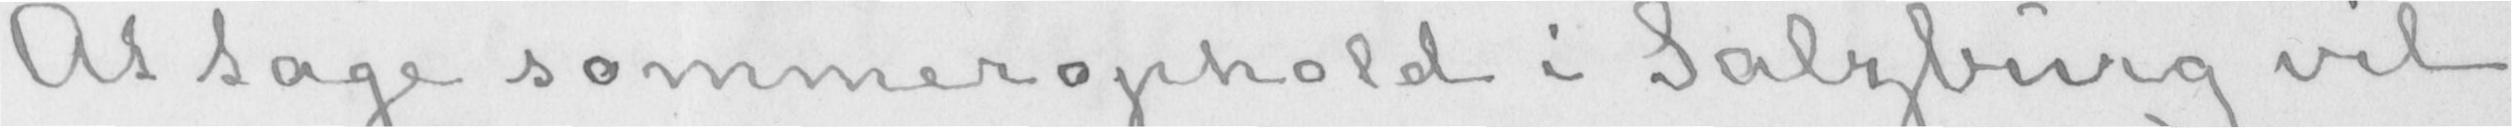At tage sommerophold i Salzburg vil
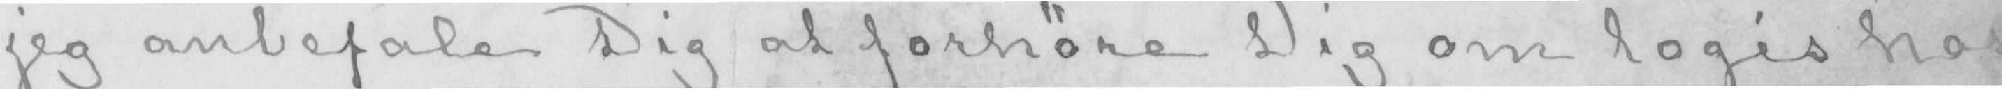jeg anbefale Dig at forhøre Dig om logis hos
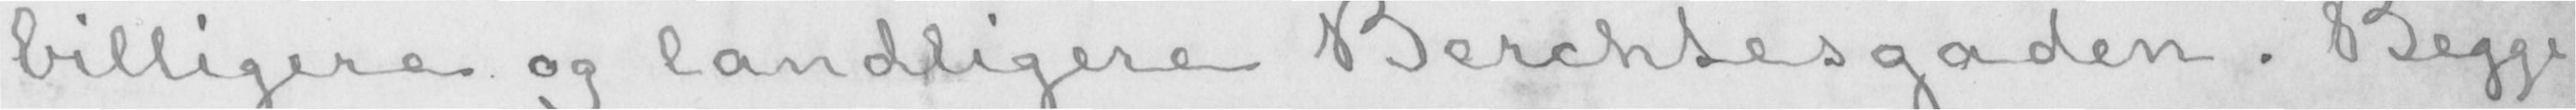billigere og landligere Berchtesgaden. Begge
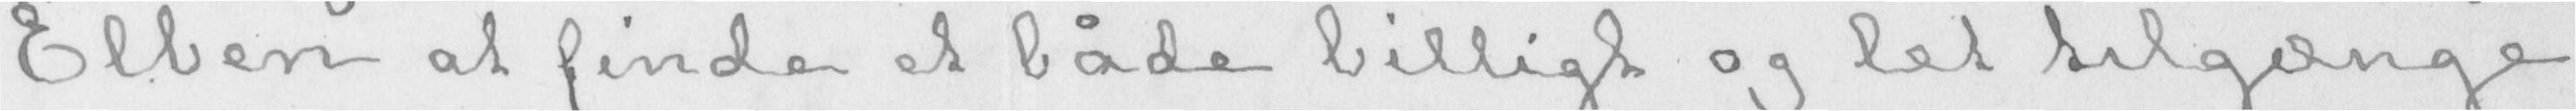Elben at finde et både billigt og let tilgænge-
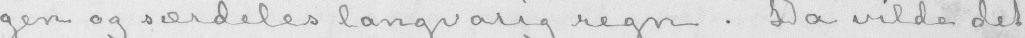gen og særdeles langvarig regn. Da vilde det
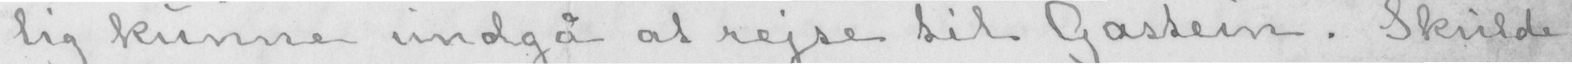lig kunne undgå at rejse til Gastein. Skulde
